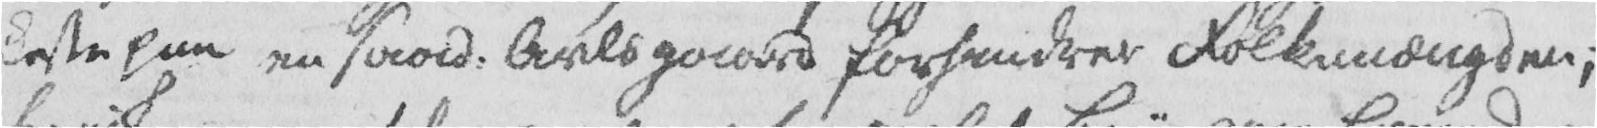koste paa en saad. Avlsgaard forhindrer Folkemængden;
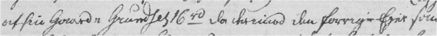af sin Gaard i Grundset 16 rd da derimod den forrige Ejer som
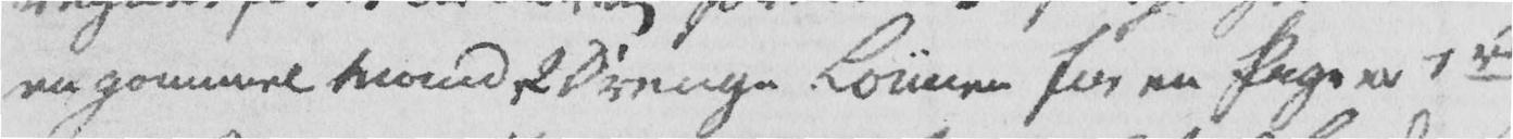en gammel Mand 2 Drenge. Lønnen for en Pige er 1 rd
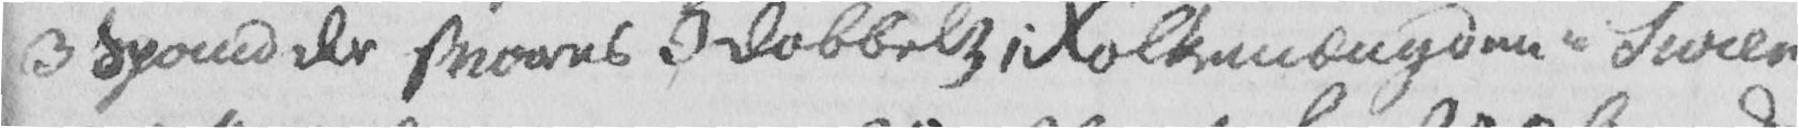3 Spand der svares 3 Dobbelt; Folkemængden i Suren
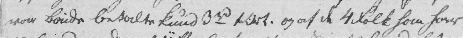var Bonde betalte kund 3 rd 1 Ort. og af de 4 Folk han have
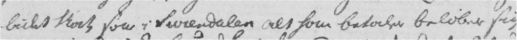lidet Skat som i Surendalen alt han betaler beløber sig
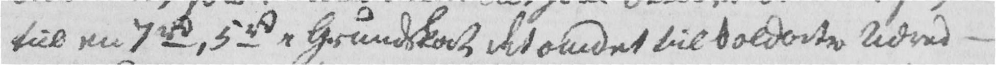til en 7 rd, 5 rd i Grundskat det andet til Soldates Udred-
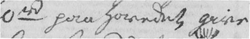0 rd paa Hovedet give
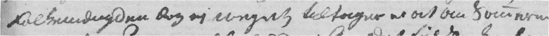Folkemængden dog ei meget tiltager er at om Someren
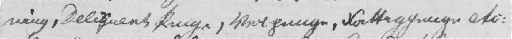ning, Deliquent Penge, Veipenge, Fattigpenge etc.
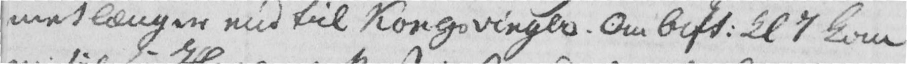met længer end til Kongsvinger. Om Aft. kl 7 kom
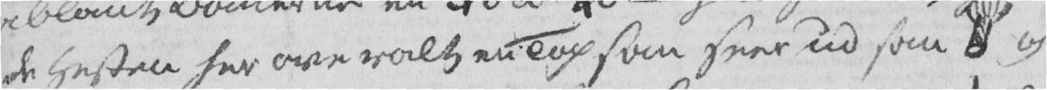de Hesten her overalt en Top som seer ud som  og
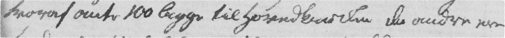hvoraf omtr 100 ligge til Hovedkirken de andre ere
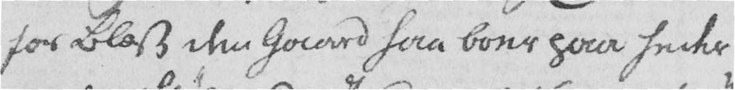for Blæst den Gaard han boer paa heder
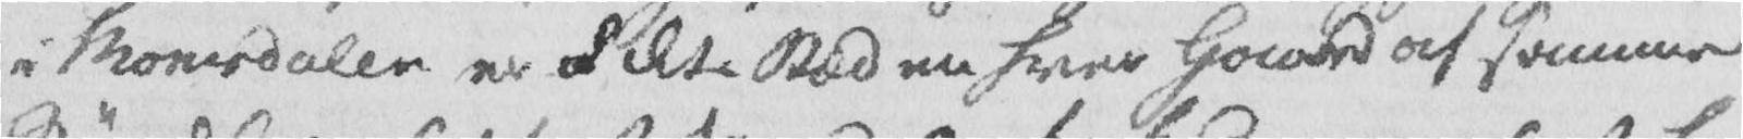i Monerdalen er i dette Stæd en hver Gaard af samme
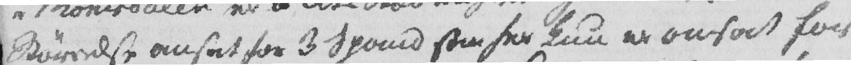Størrelse ansat for 3 Spand som her kun er ansat for
